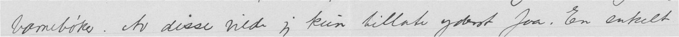barnebøker. Av disse vilde jeg kun tillate yderst faa. En enkelt
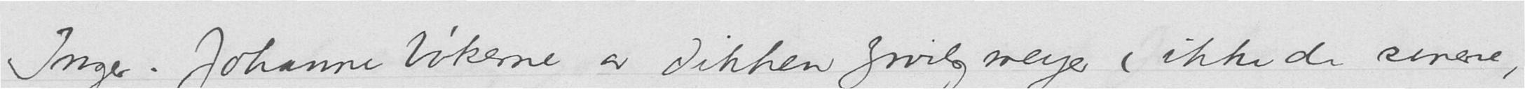Inger-Johanne bøkerne av Dikken Zwilgmeyer (ikke de senere,
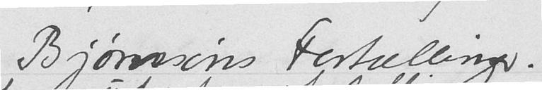Bjørnsons Fortællinger.
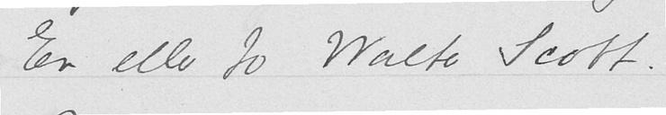En eller to Walter Scott.
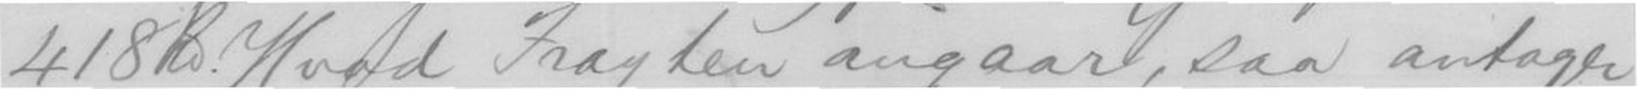418 Rd. Hvad Fragten angaar, saa antager
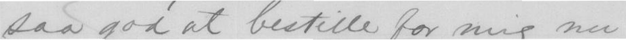saa god at bestille for mig nu
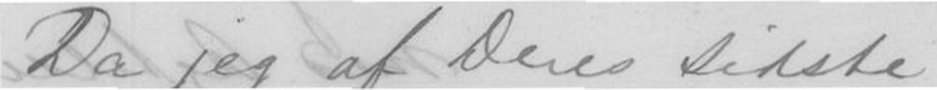Da jeg af Deres sidste
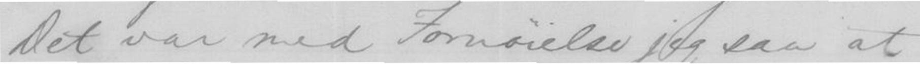Det var med Fornøielse jeg saa at
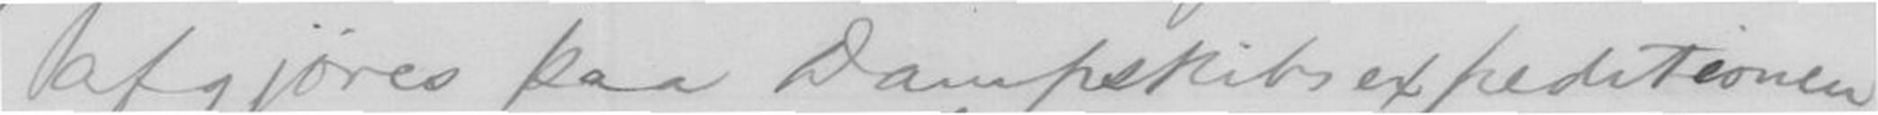af gjøres paa Dampskibs expedetionen
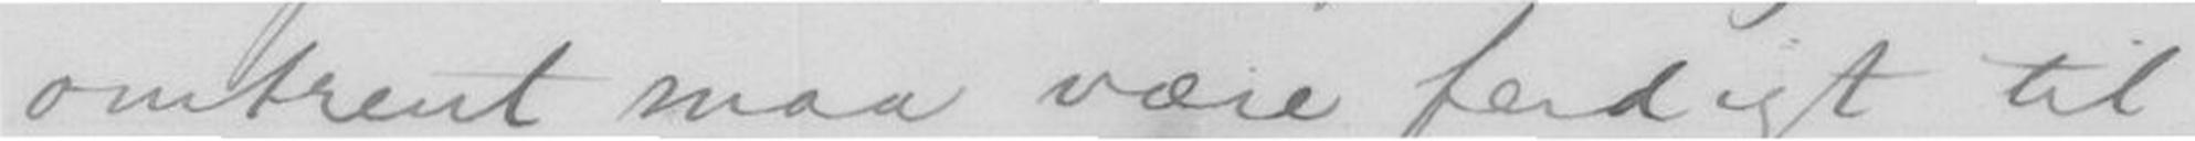omtrent maa være ferdigt til
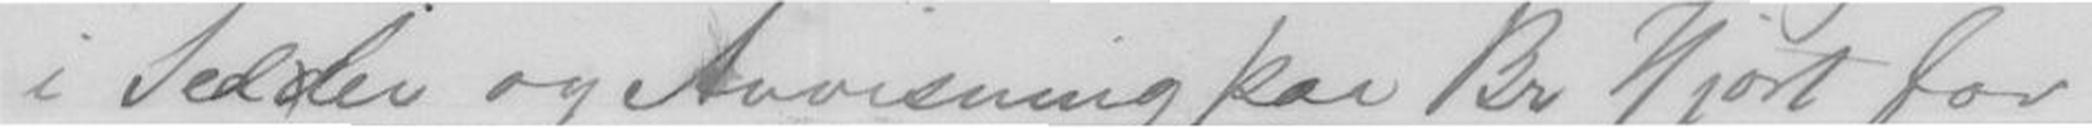i Sedler og Anvisning paa Br Hjort for
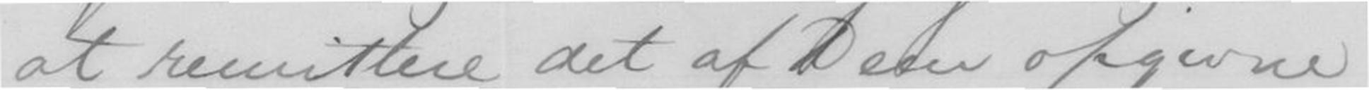at remitere det af Dem opgivne
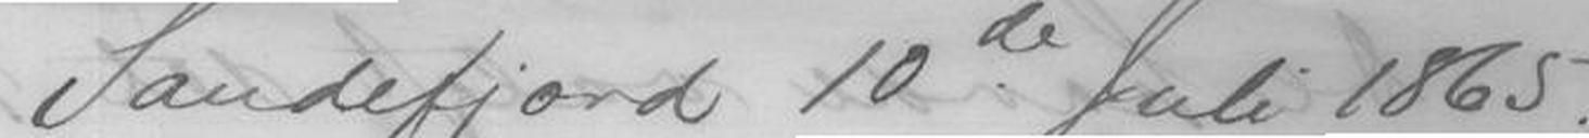Sandefjord 10de Juli 1865.
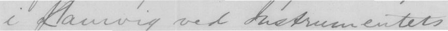i Laurvig ved Instrumentets
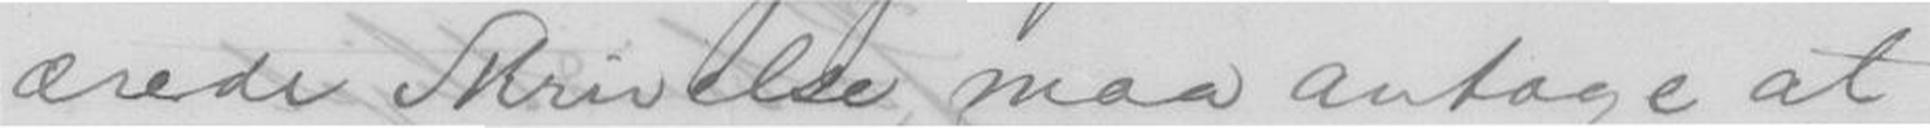ærede Skrivelse maa antage at
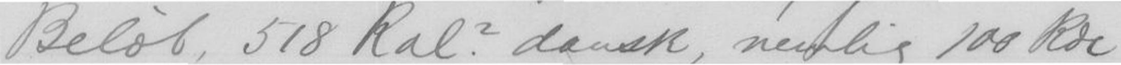Beløb, 518 Ral? dansk, nemlig 100 Rdl
